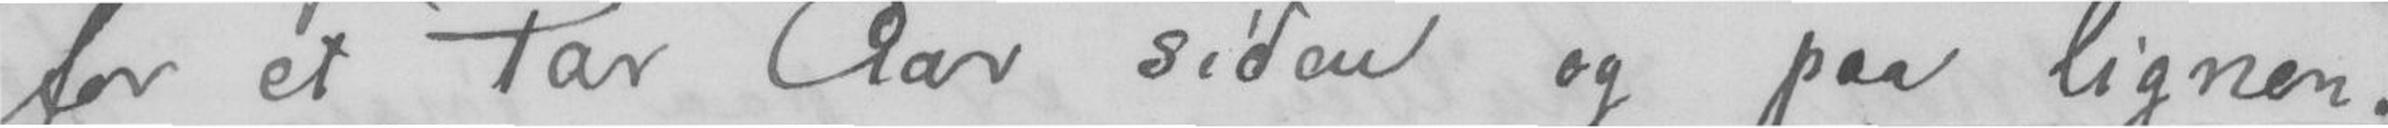for et Par Aar siden og paa lignen-
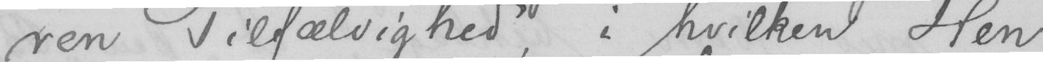ren Tilfældighed, i hvilken Hen
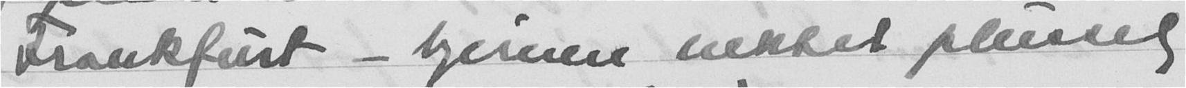Frankfurt - hjernen nektet plutselig
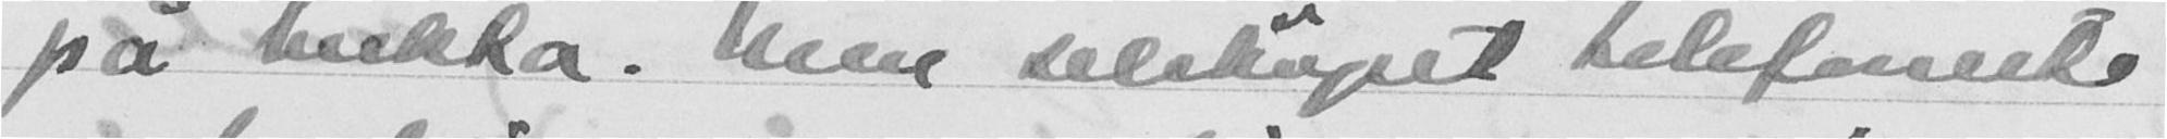på bukta. Men selskapet telefonerte
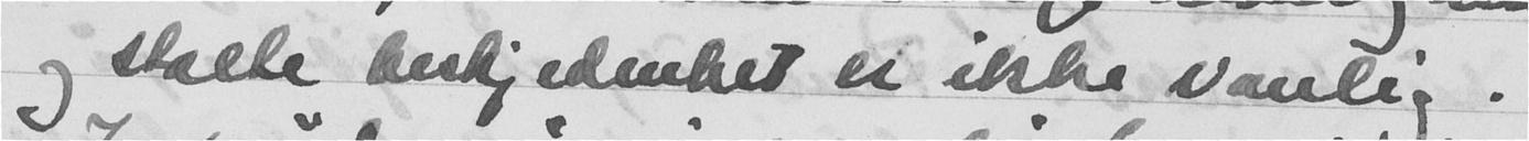og stolte beskjedenhet er ikke vanlig.
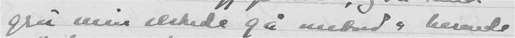gru min elskede gå ombords hernede
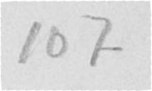107
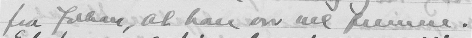fra Johan, at han var vel fremme.
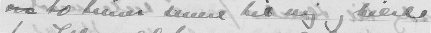to timer senere til mig, hilste
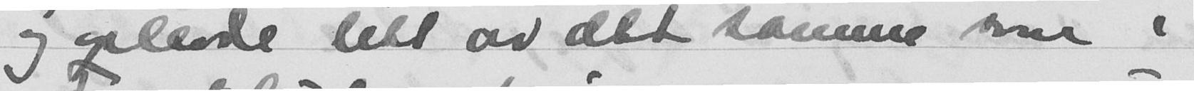og oplevde litt av det samme som i
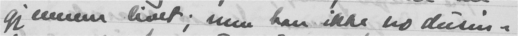gjennom livet; men han ikke no dusin-
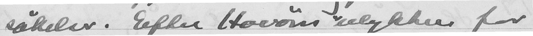søkelser. Efter Havørn ulykken for
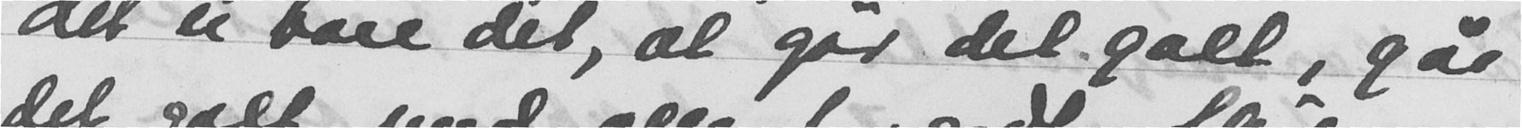det er bare det, at går det galt, går
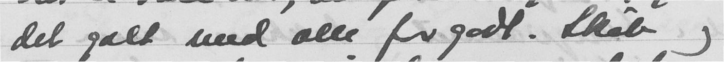det galt med alle for godt. Skib og
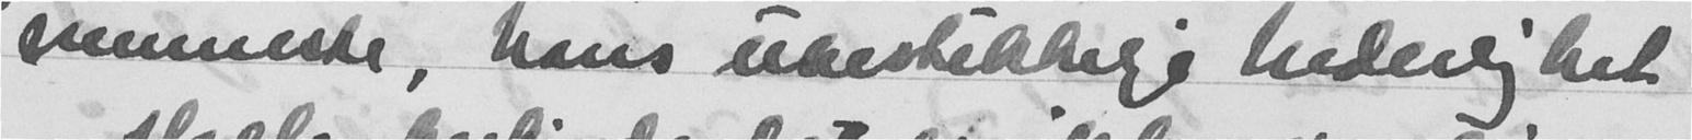menneske, hans ubestikkelige hederlighet
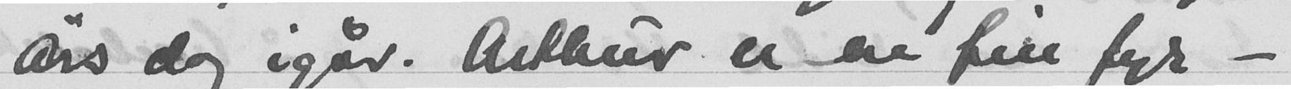års dag igår. Arthur er en fin fyr -
